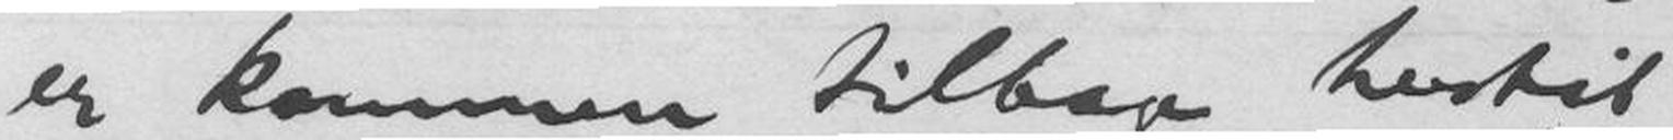er kommen tilbage hertil
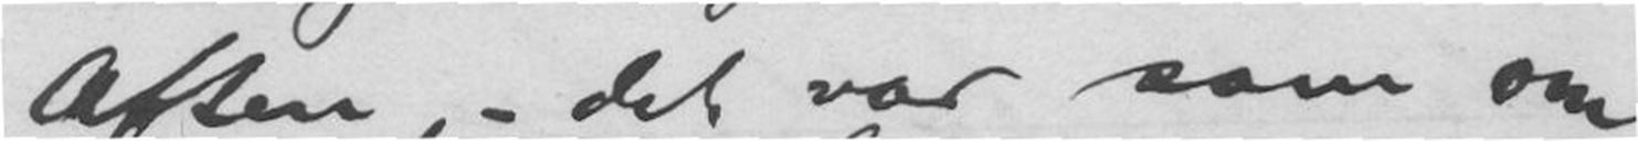Aften, - det var som om
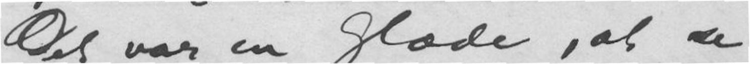Det var en Glæde, at se
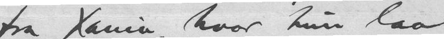fra Xania, hvor hun laa
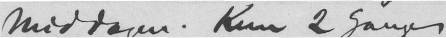Middagen. Kun 2 Ganger
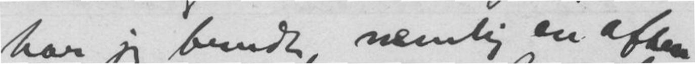har jeg brudt, nemlig en Aften
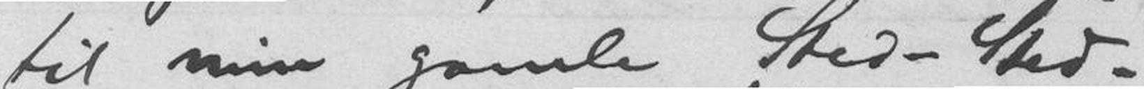til min gamle Sted-Sted-
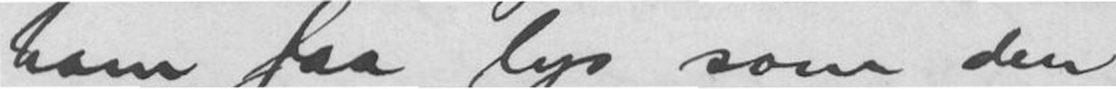ham saa lys som den
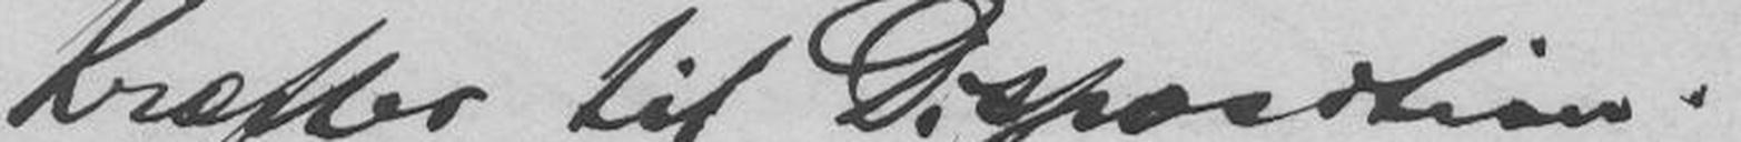Kræfter til Disposition. Jeg
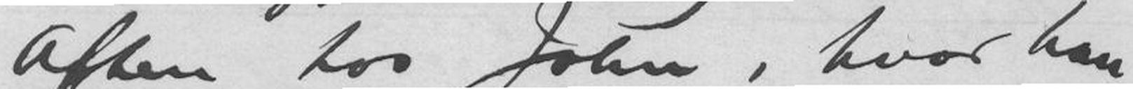Aften hos John, hvor han
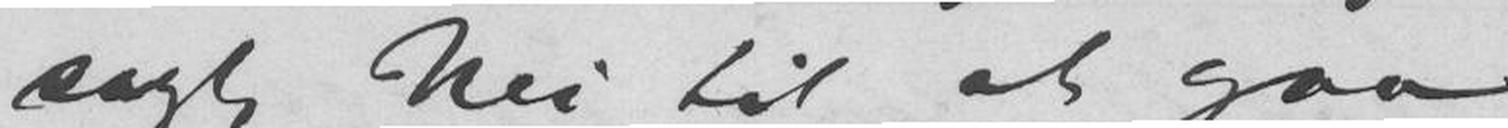sagt Nei til at gaa
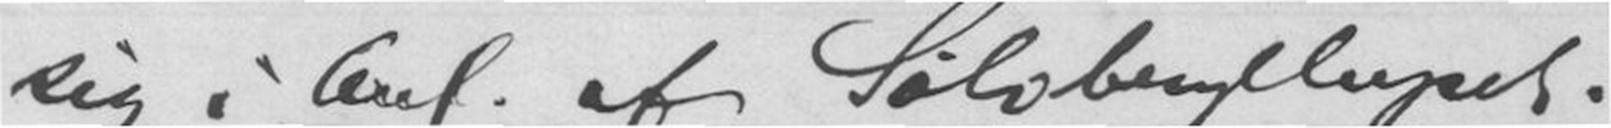sig i Anl. af Sølvbryllupet.
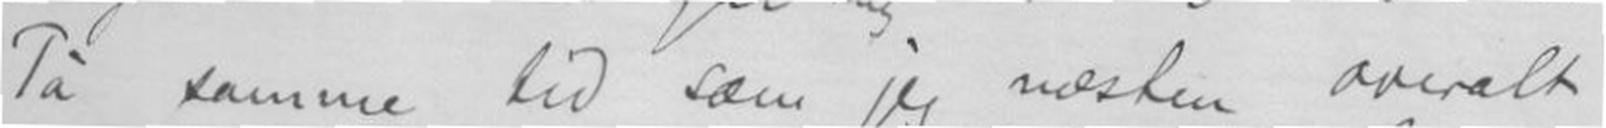På samme tid som jeg næsten overalt
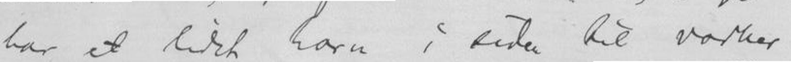har et lidet horn i siden til vorher
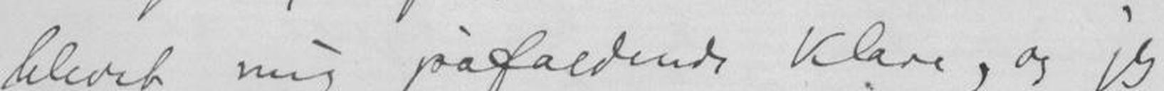blevet mig påfaldende klare, og jeg
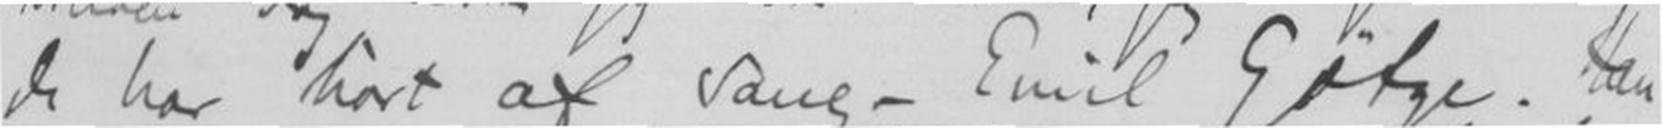de har hørt af sang - Emil Götge. Han
